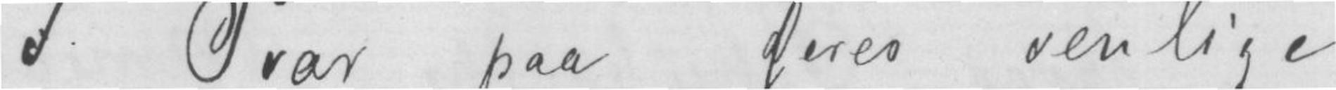I Svar paa Deres venlige
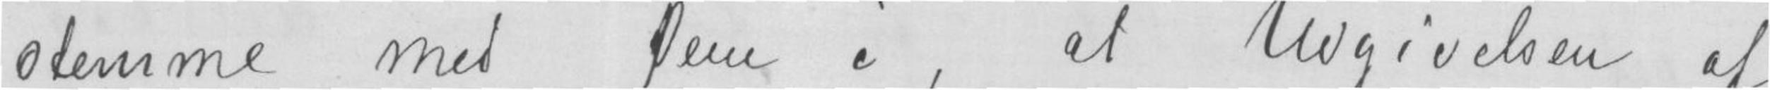stemme med Dem i, at Udgivelsen af
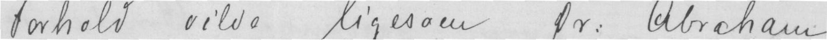Forhold vilde ligesom Dr. Abraham
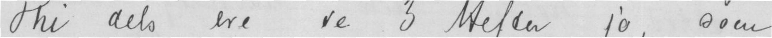Thi dels ere de 3 Hefter jo, som
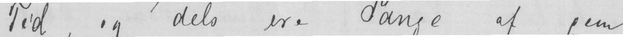Tid, og dels ere Sange af dem
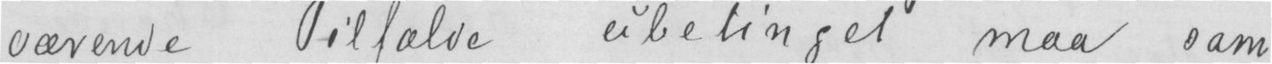værende Tilfælde ubetinget maa sam-
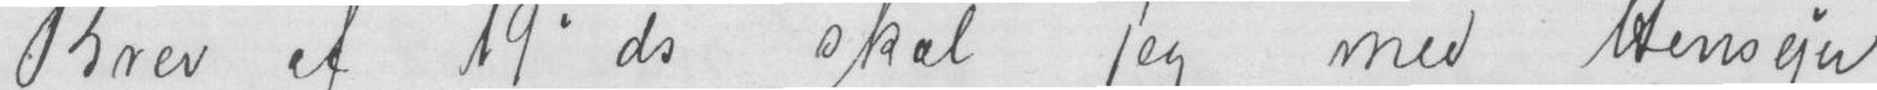Brev af 19 ds skal jeg med Hensyn
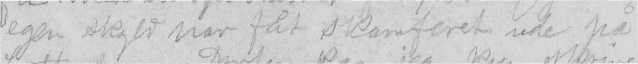egen skyld har fåt skamferet ude på
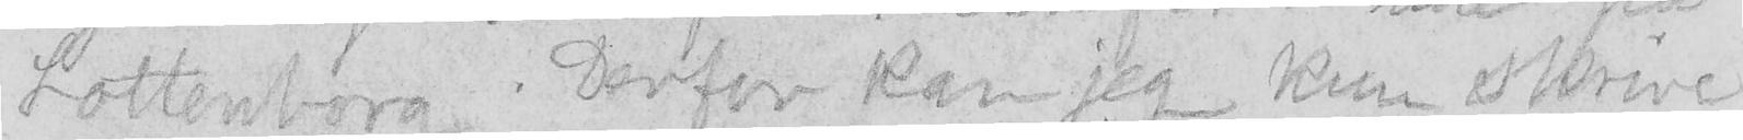Lottenborg. Derfor kan jeg kun skrive
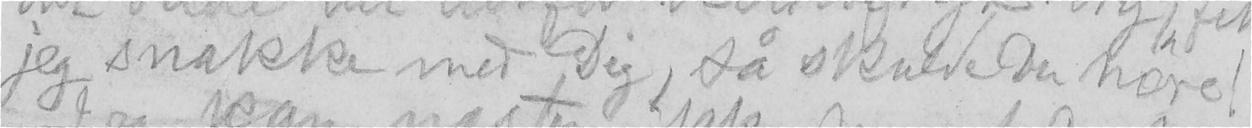jeg snakke med Dig, så skulde Du høre!
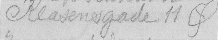Klasensgade 11 Ø
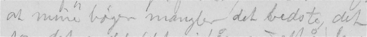at mine bøger mangler det bedste, det
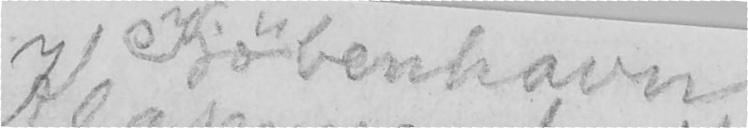Kjøbenhavn
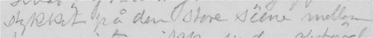stykket på den store scene mellem
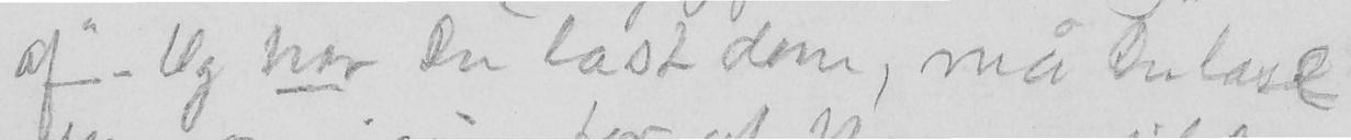af". Og har Du læst dem, må Du læse
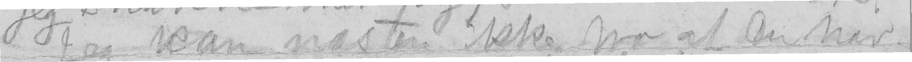Jeg kan næsten ikke tro at Du har
